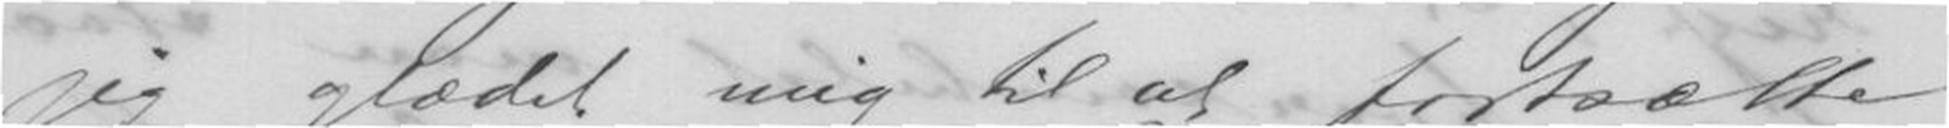jeg glædet mig til at fortsætte,
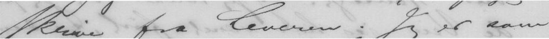skrive fra Leveren. Jeg er som
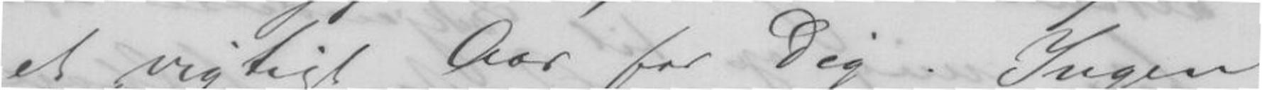et vigtig Aar for Dig. Ingen
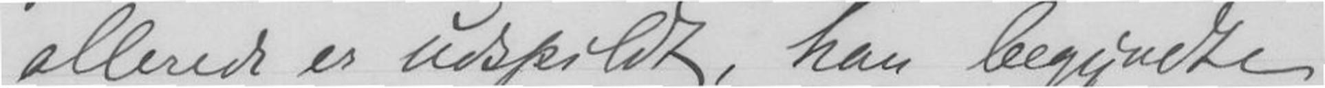allerede er udspildt, han begyndte
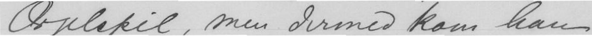Orgelspil, men dermed kom han
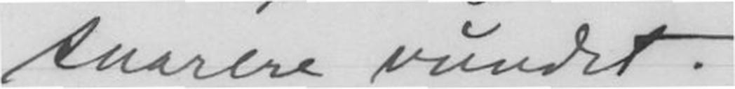snarere vundet.
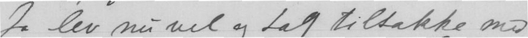Ja lev nu vel og tag tiltakke med
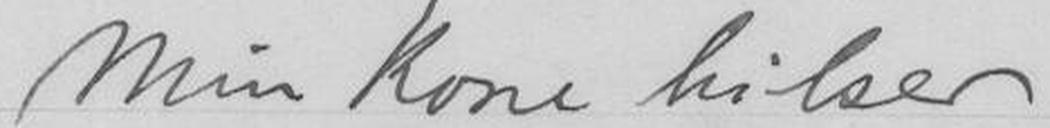Min Kone hilser!
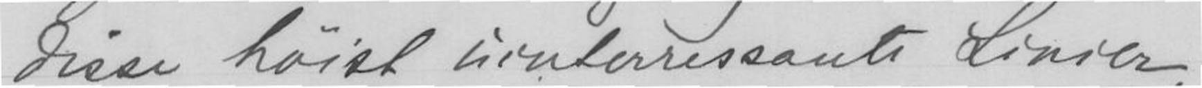disse høist uinterressante Linier
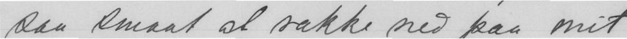saa smaat at rakke med paa mit
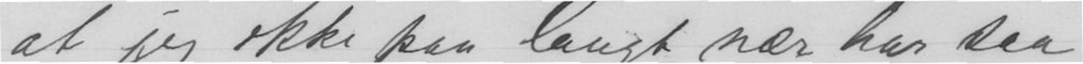at jeg ikke paa langt nær har saa
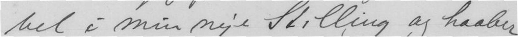vel i min nye Stilling og haaber
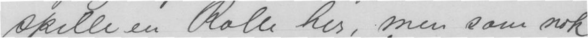spille en Rolle her, men som nok
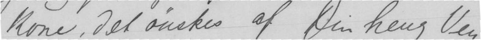Kone, det ønskes af Din heng Ven
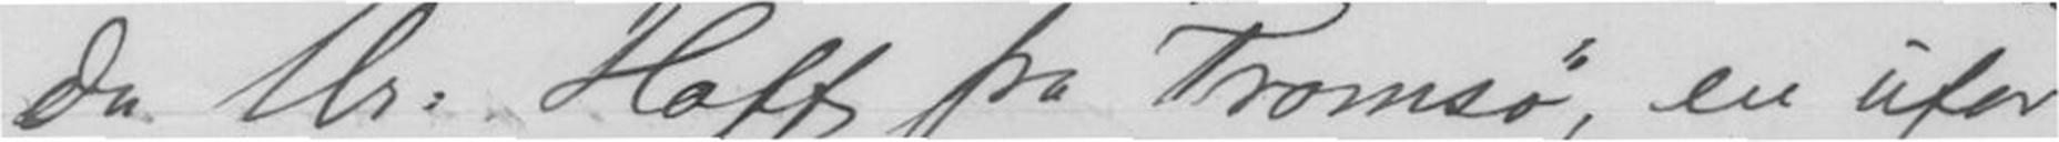da Hr: Hoff fra Tromsø, en ufor
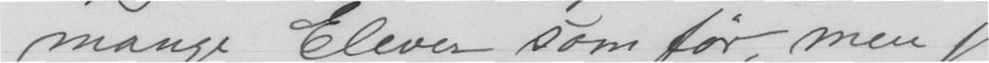mange Elever som før, men jeg
